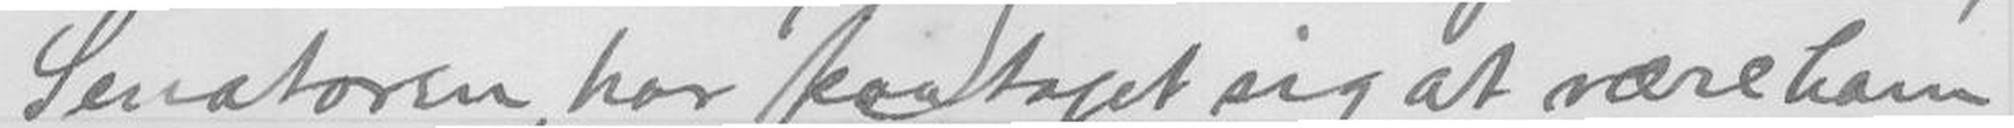Senatoren, har paataget sig at være ham
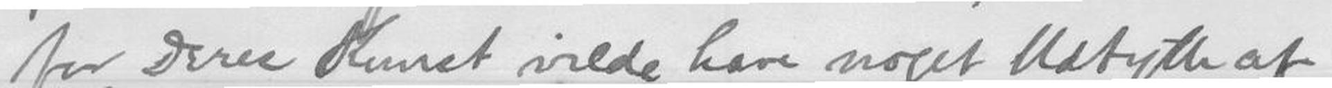De for Deres Kunst vilde have noget Udbytte af
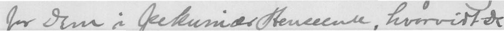for Dem i pekuniær Henseende, hvorvidt
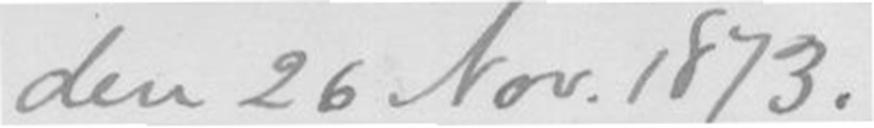den 26 Nov. 1873.
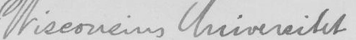Wisconcins Universitet
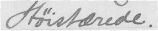Høistærede.
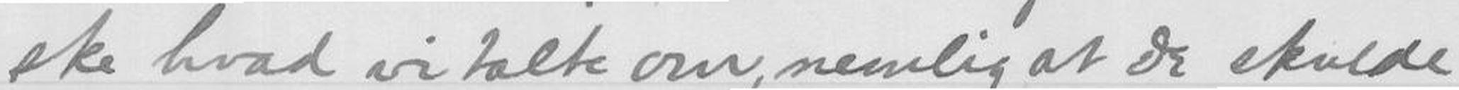ske hvad vi talte om, nemlig at De skulde
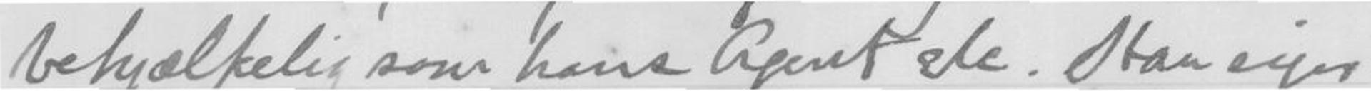behjælpelig som hans Agent etc. Han siger
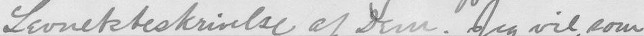Levnekskrivelse af Dem. Jeg vil, som
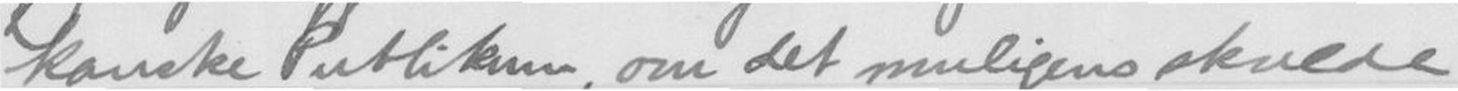kanske Publikum, om det muligens skulde
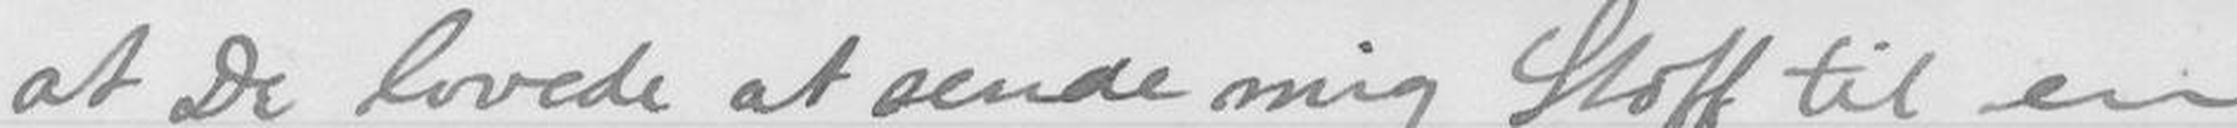at De lovede at sende mig Stoff til en
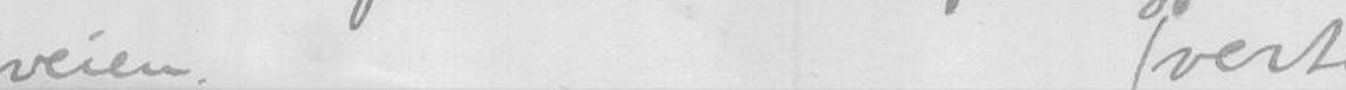iveien(verte)
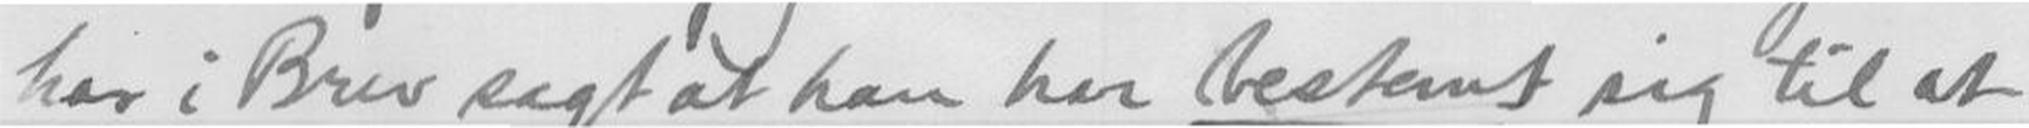har i Brev sagt at han har bestemt sig til at
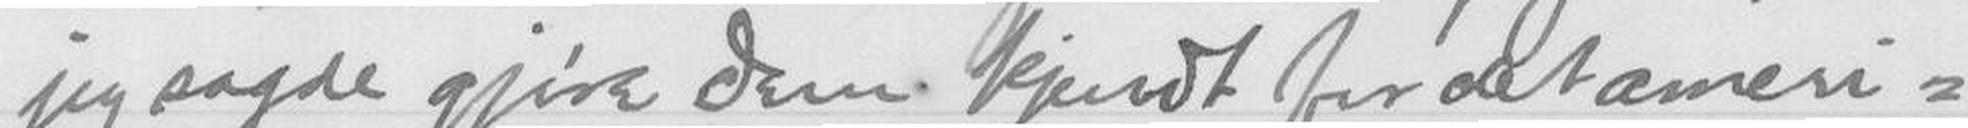jeg sagde gjøre Dem kjendt for det ameri-
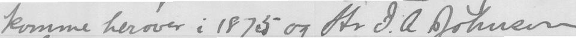komme herover i 1875 og Hr I.A. Johnsen,
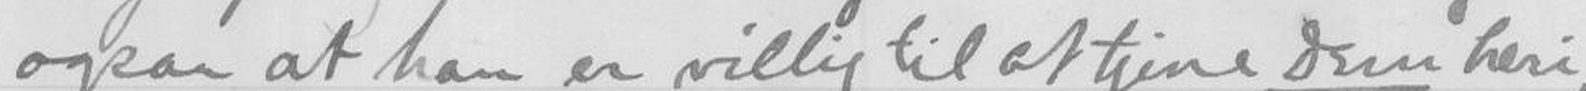ogsaa at han er villig til at tjene Dem heri,
